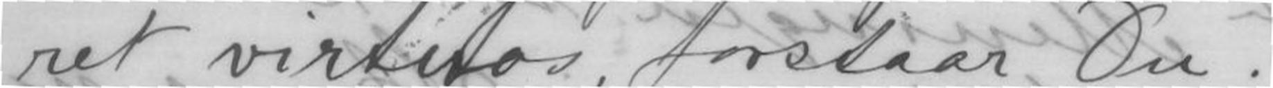ret virtuos, forstaar Du.
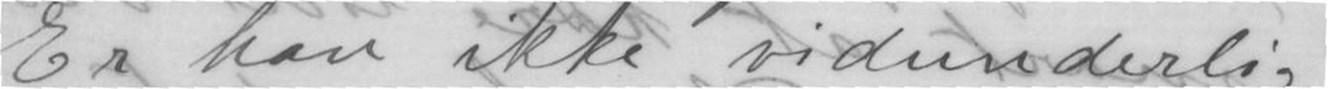Er han ikke vidunderlig?
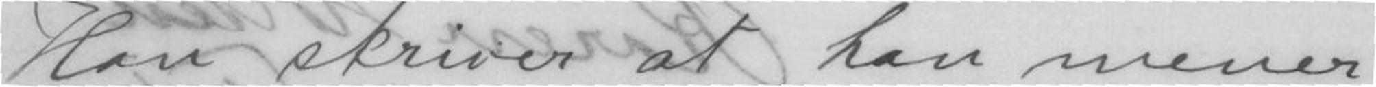Han skriver at han mener
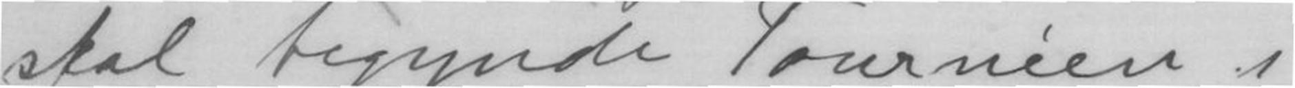skal begynde Tournéen i
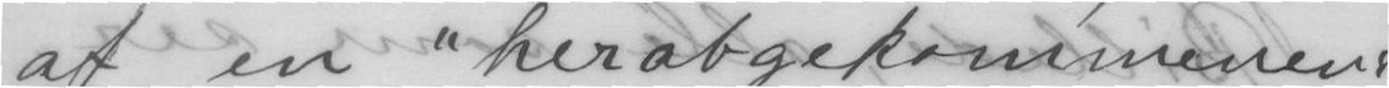af en "herabgekommenen"
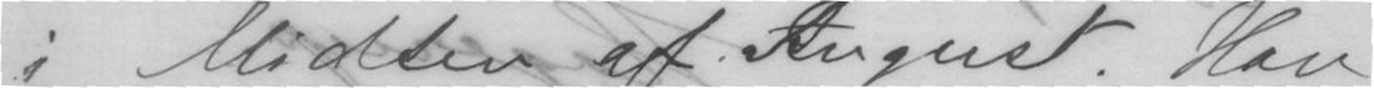i midten af August. Han
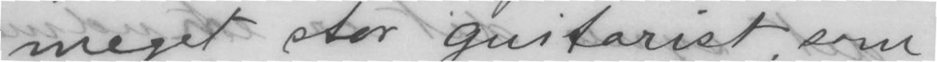meget stor guitarist, som
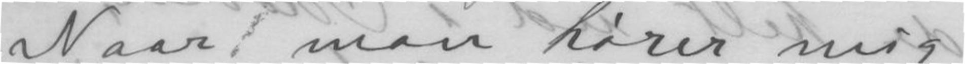Naar man hører mig
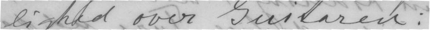lighed over Guitaren.
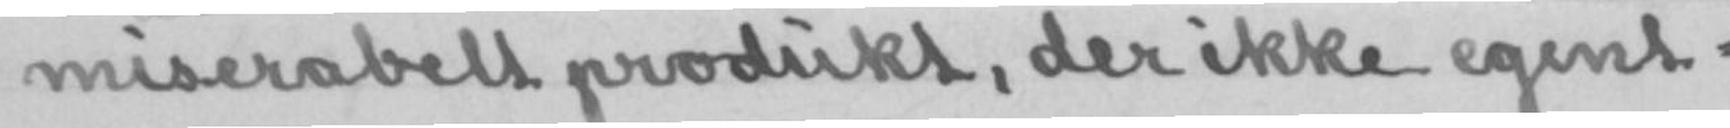miserabelt produkt, der ikke egent-
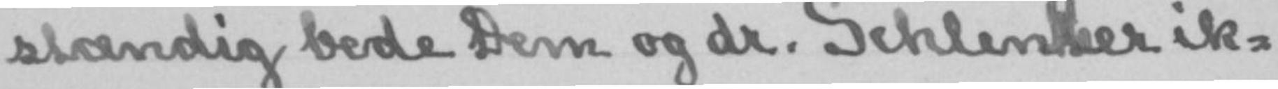stændig bede Dem og dr. Schlenther ik-
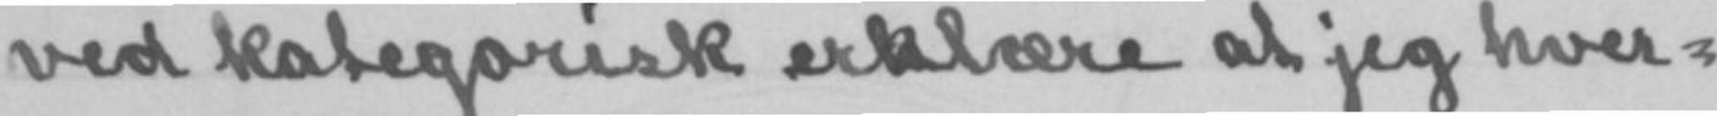ved kategorisk erklære at jeg hver-
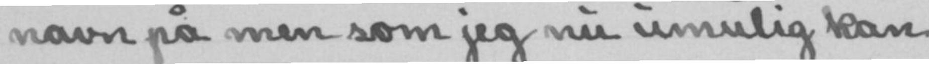navn på men som jeg nu umulig kan
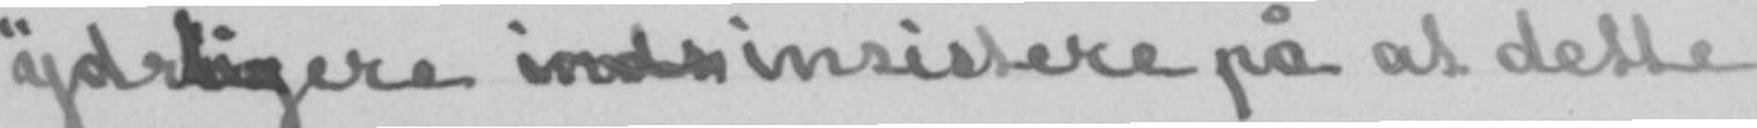ke ydrligere inds insistere på at dette
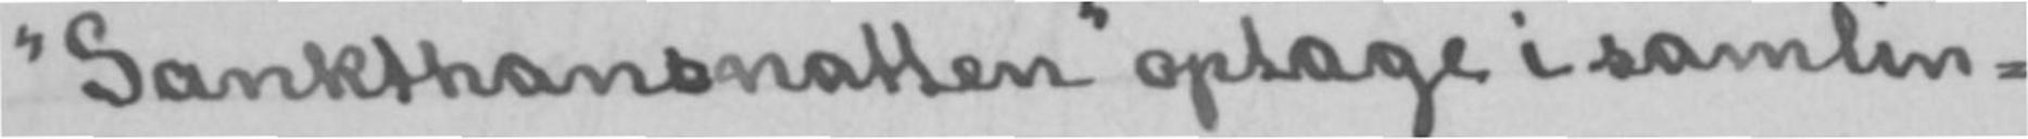«Sankthansnatten» optage i samlin-
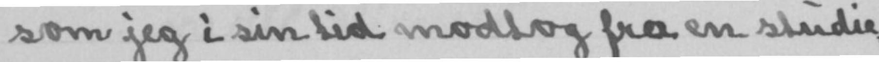som jeg i sin tid modtog fra en studie-
